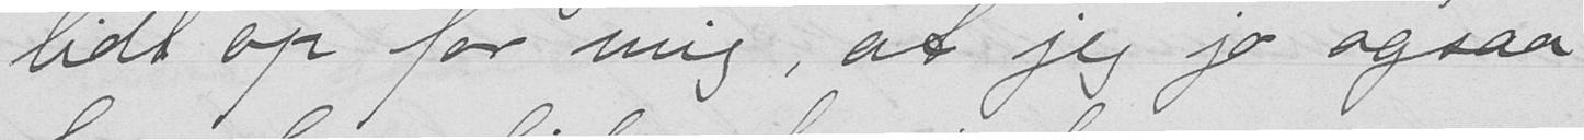lidt op for mig, at jeg jo ogsaa
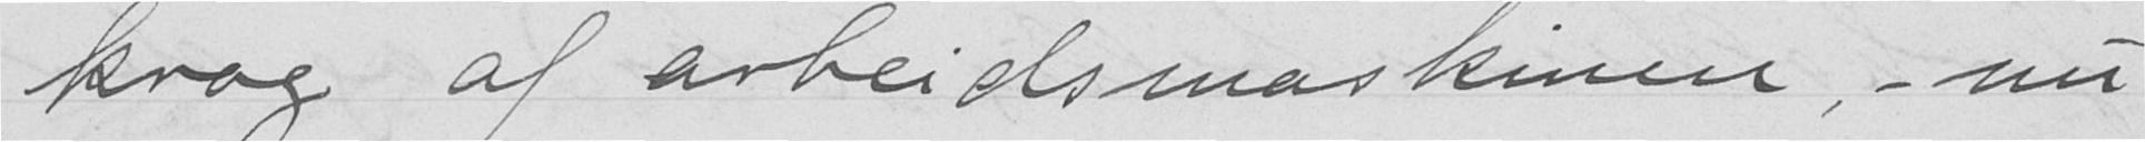krog af arbeidsmaskinen, - nu
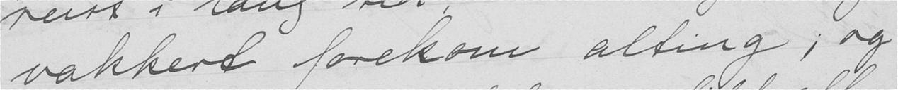vakkert forekom alting, og
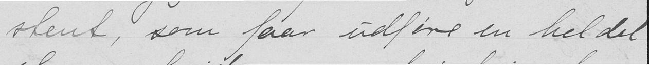stent, som faar udføre en hel del
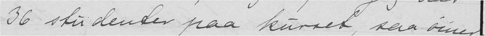36 studenter paa kurset, saa øiner
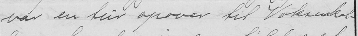var en tur opover til Voksenkol-
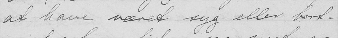at have været syg eller bort-
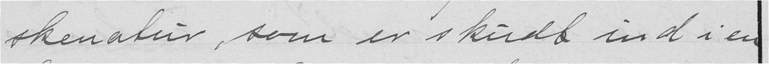skenatur, som er skudt ind i en
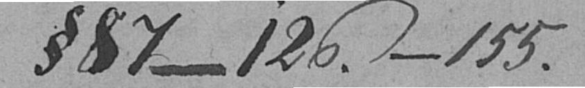§ 87-126.-155.
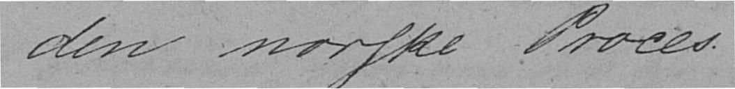den norske Proces.
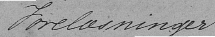Forelæsninger
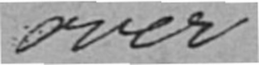over
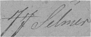JJ Selmer
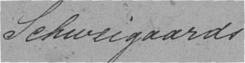Schweigaards
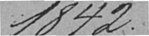1842.
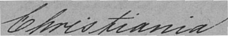Christiania
